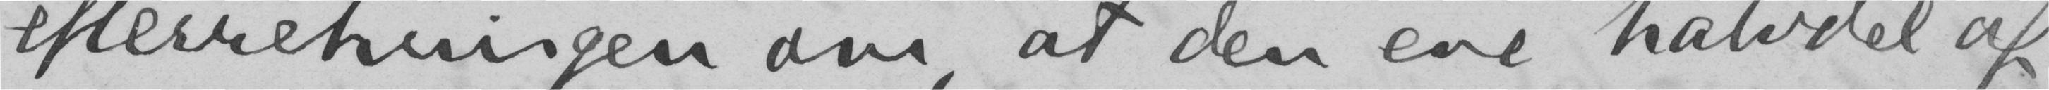efterretningen om, at den ene halvdel af
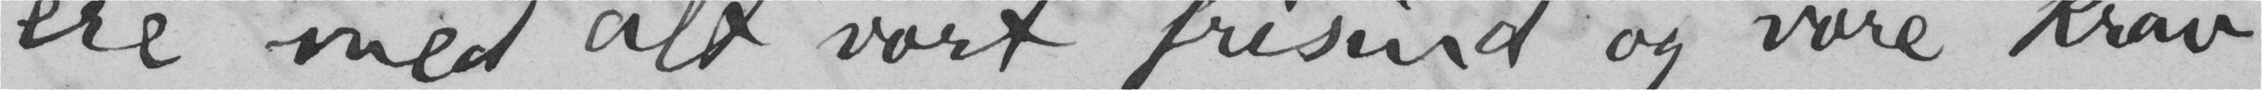ere med alt vort frisind og vore krav
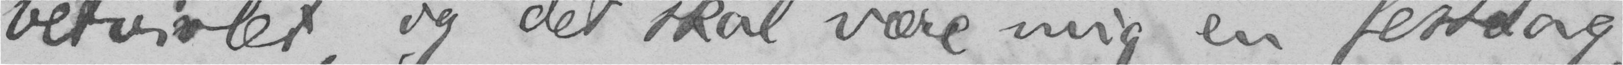betvivlet, og det skal være mig en festdag
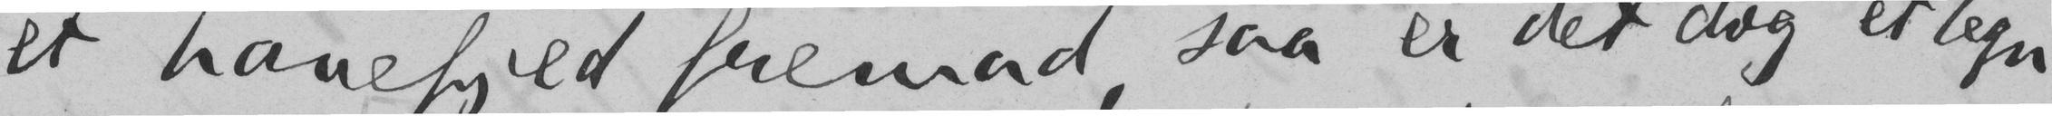et hanefjed fremad, saa er det dog et tegn
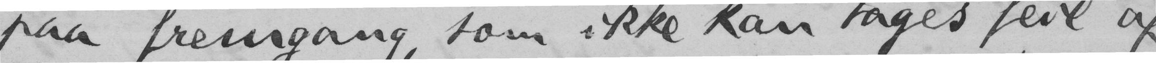paa fremgang, som ikke kan tages feil af.
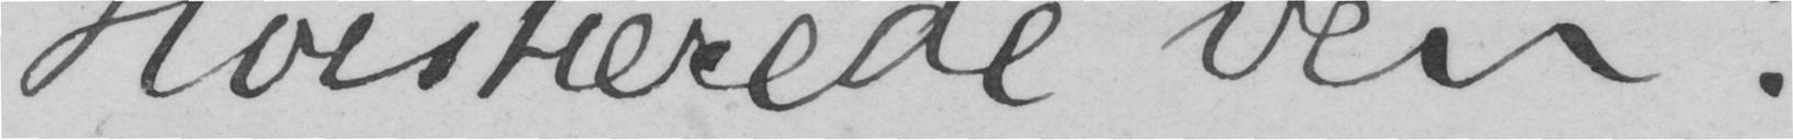Høistærede ven!
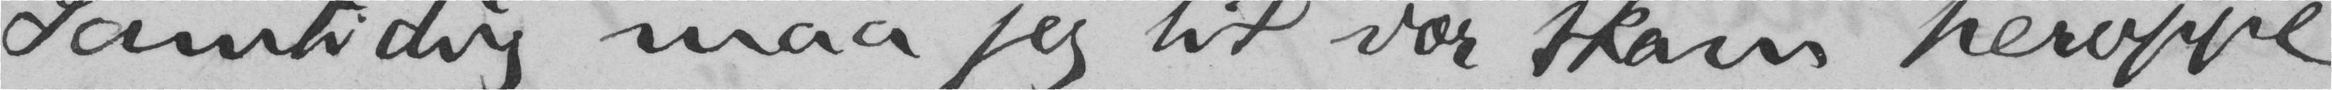Samtidig maa jeg til vor skam heroppe
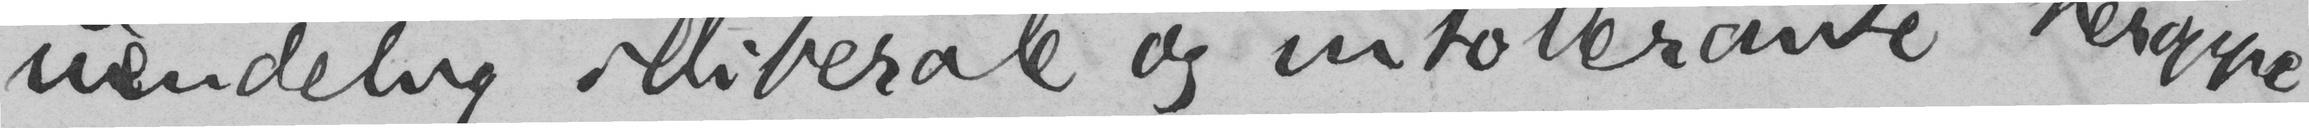uendelig illiberale og intollerante heroppe.
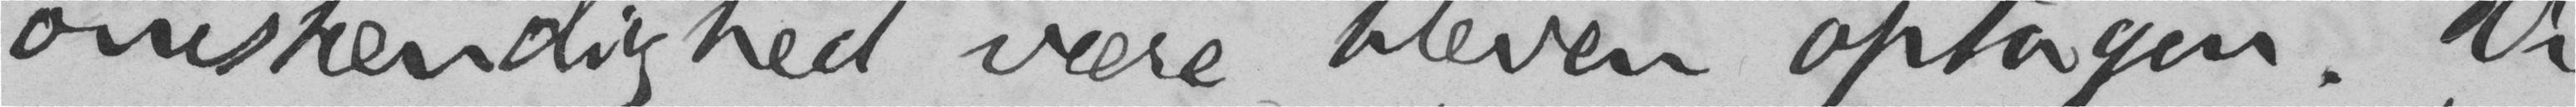omstændighed være bleven optagen. Vi
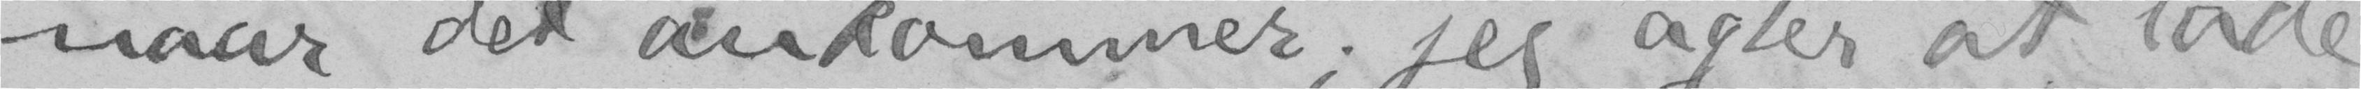naar det ankommer; jeg agter at lade
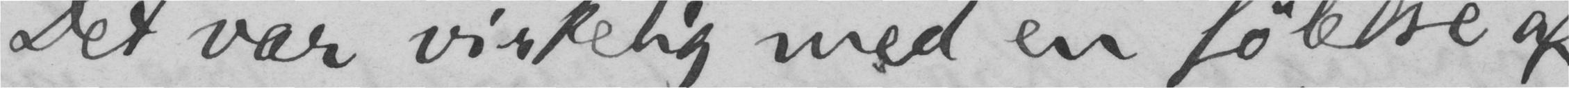Det var virkelig med en følelse af
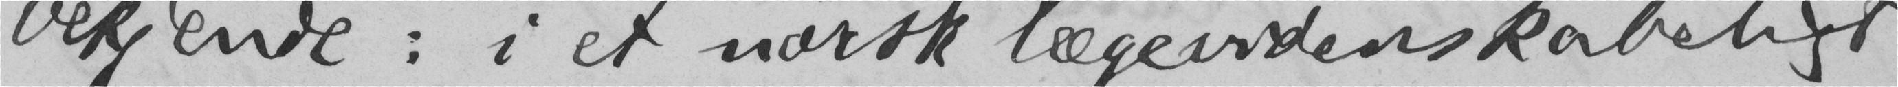bekjende: i et norsk lægevidenskabeligt
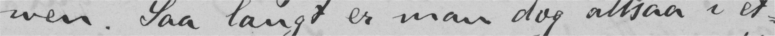men. Saa langt er man dog altsaa i et-
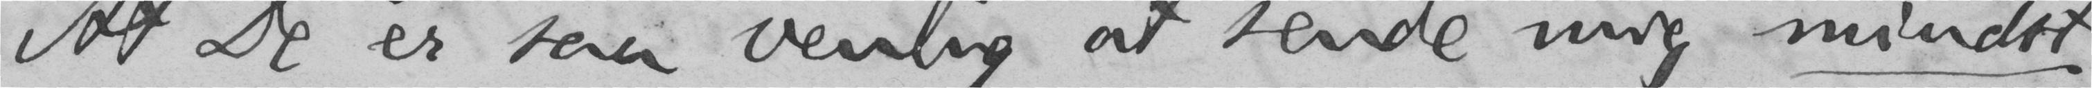At De er saa venlig at sende mig mindst
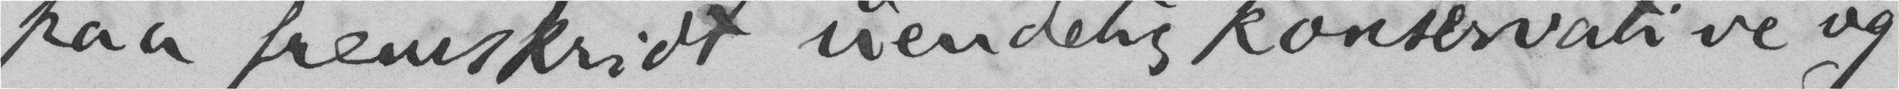paa fremskridt uendelig konservative og
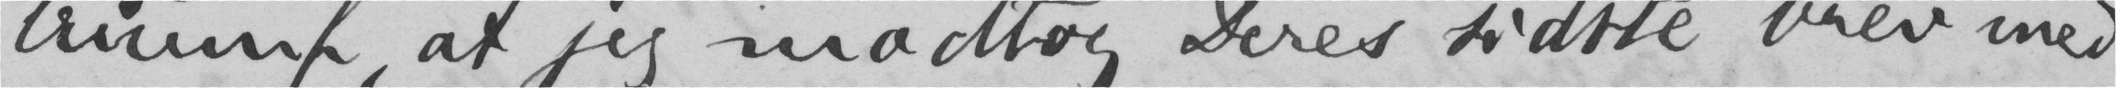triumf, at jeg modtog Deres sidste brev med
